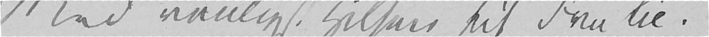Med venligst Hilsen til Fru Lie.
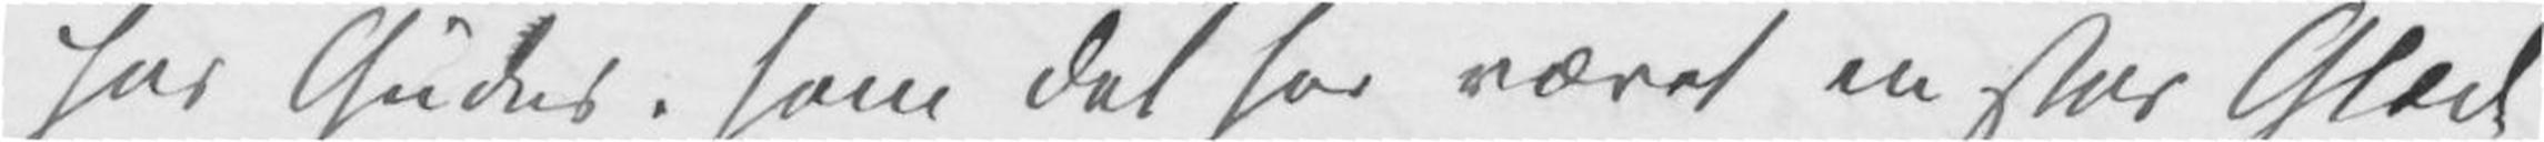hos Gudes, som det har været en stor Glæde
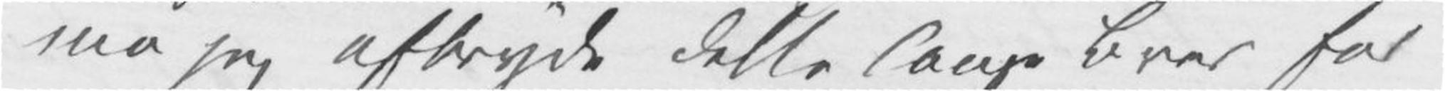må jeg afbryde dette lange Brev for
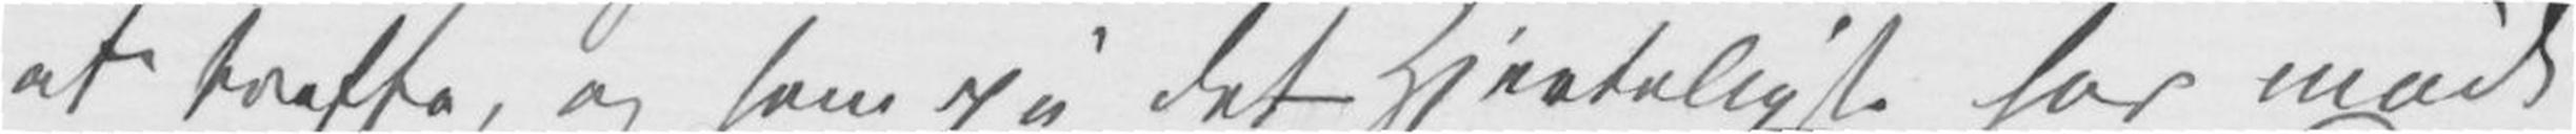at treffe, og som på det hjerteligste har mødt
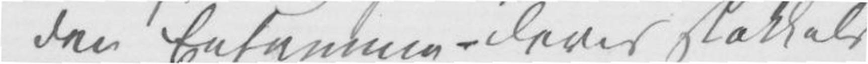den Ensomme – Deres stakkels
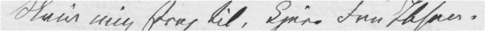Skriv mig strax til, kjere Fru Ibsen,
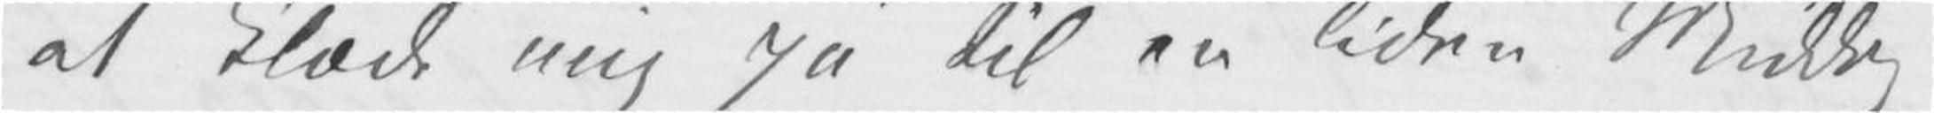at klæde mig på til en liden Middag
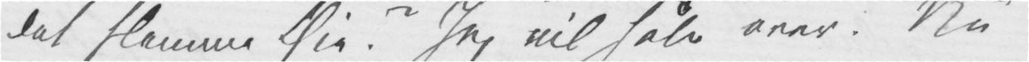det slemme Øie? Jeg vil håbe over. Nu
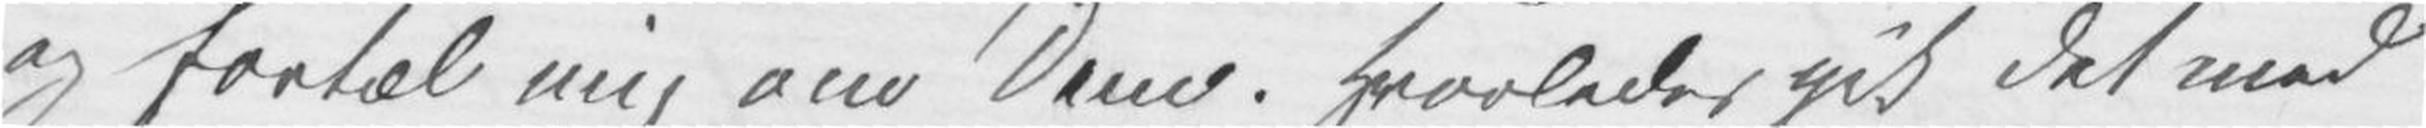og fortæl mig om Dem. Hvorledes gik det med
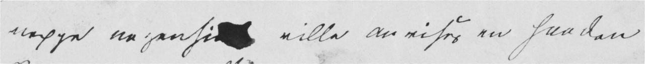neppe nogensinde ville anvises en saadan
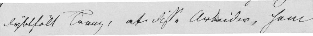dybtfølt Trang, at disse Arbeider, som
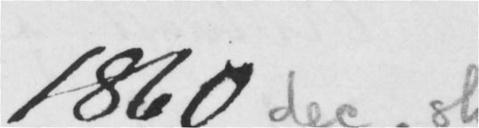1860
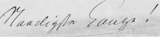Naadigste Konge!
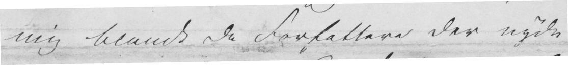mig blandt de Forfattere der nyde
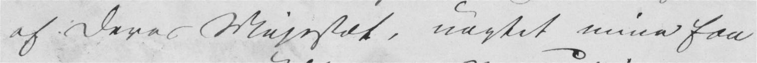af Deres Majestæt, uagtet mine faa
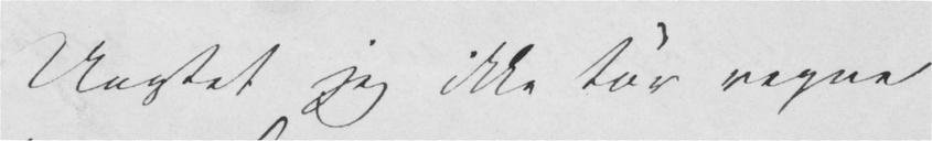Uagtet jeg ikke tør regne
